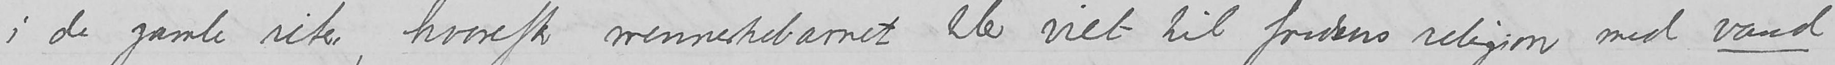i de gamle riter, hvorefter menneskebarnet ble viet til fredens religion med
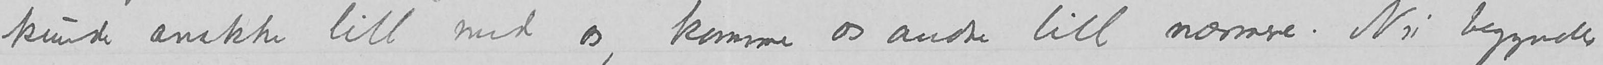kunde snakke litt med os, komme os andre litt nærmere.  Nu begynder
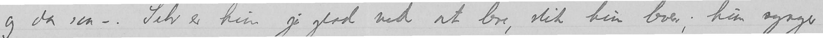og da saa – .  Selv er hun jo glad ved at leve, slik hun lever; hun synger
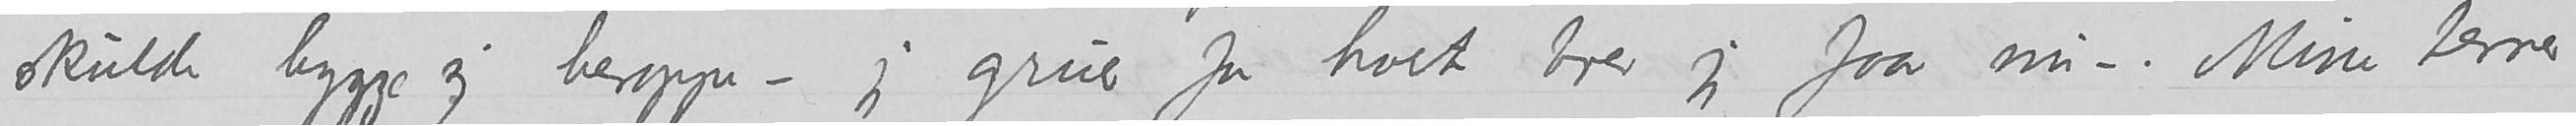skulde hygge sig heroppe – jeg gruer for hvert brev jeg faar nu –.  Mine terner
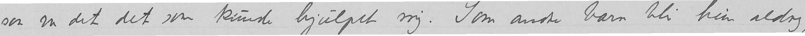saa var det det som kunde hjulpet mig.  Som andre barn bli hun aldrig,
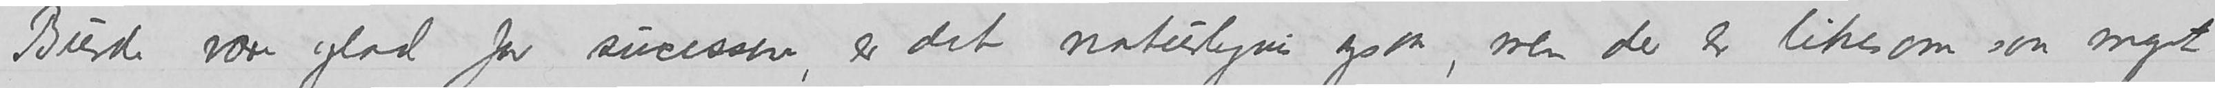Burde være glad for sucessen, er det naturligvis ogsaa, men det er likesom saa meget
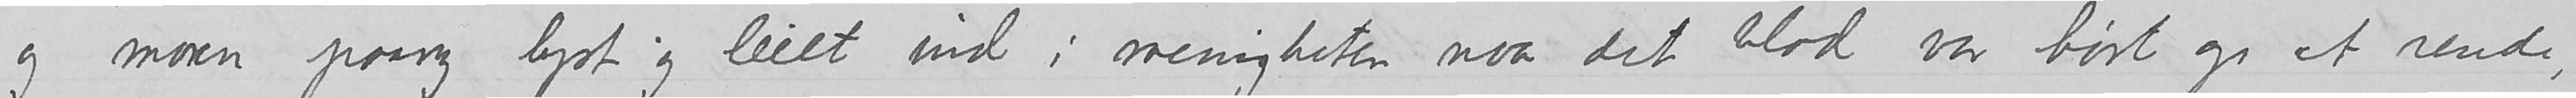vand og moren paany lyst og leiet ind i menigheten naar det blod var hørt op at rende
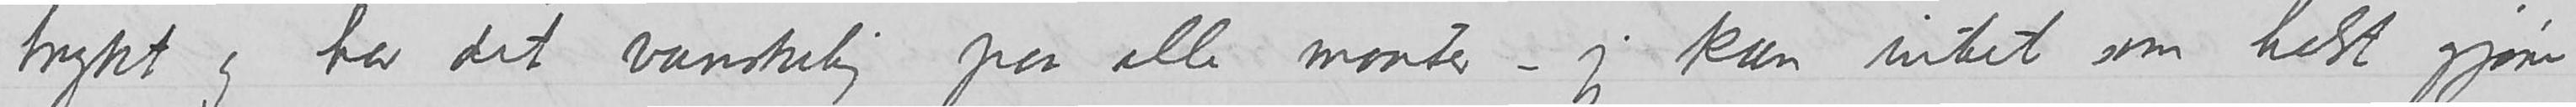trykt og har det vanskelig paa alle maater – jeg kan intet som helst gjøre
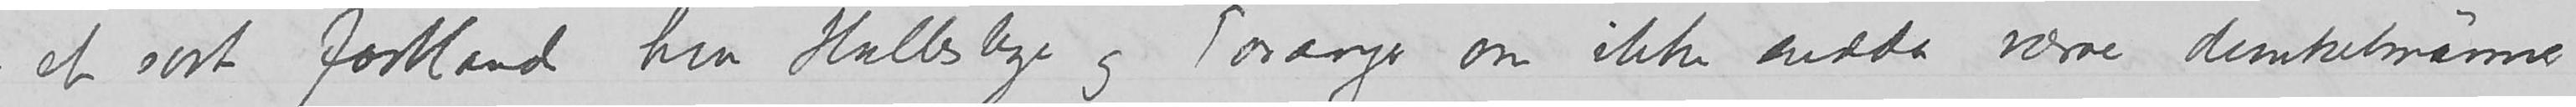– et sort fastland hvor Hallesbye og Taranger om ikke endda værre dunkelmänner
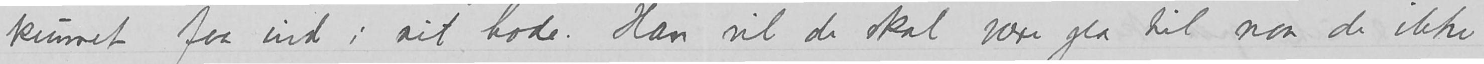kunnet faa ind i sit hode.  Han vil de skal være gla til naar de ikke
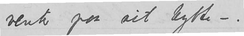venter paa sit bytte –.
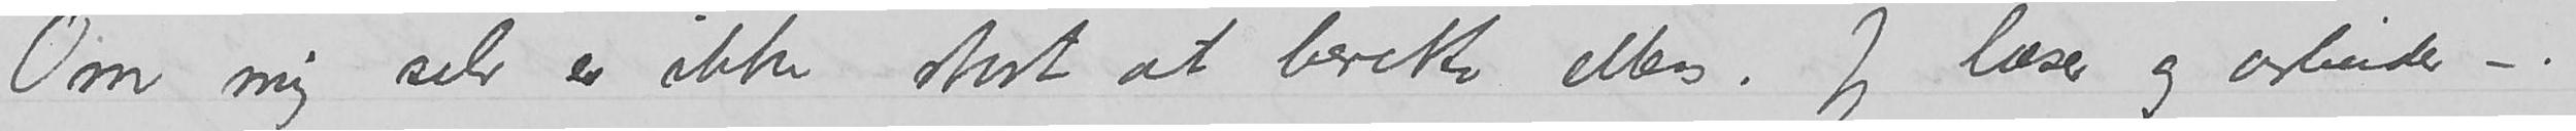Om mig selv er ikke stort at berette ellers.  Jeg læser og arbeider –.
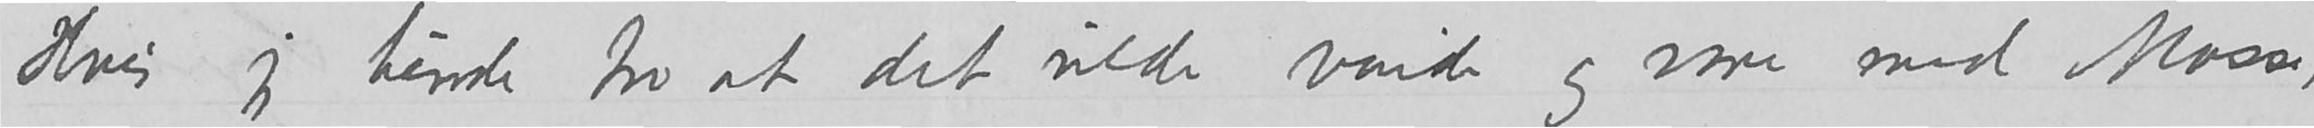Hvis jeg turde  tro at det vilde vonde og vare med Mosse,
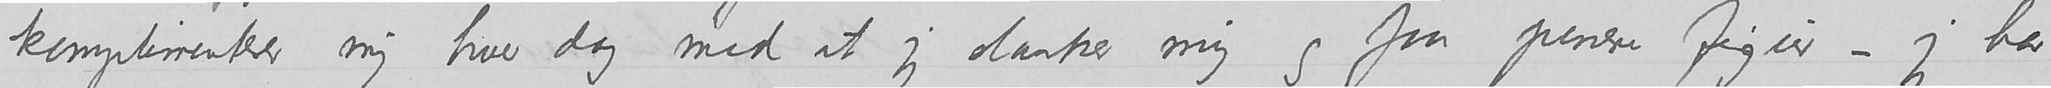komplimenterer mig hver dag med at jeg slanker mig og faar penere figur –  jeg har
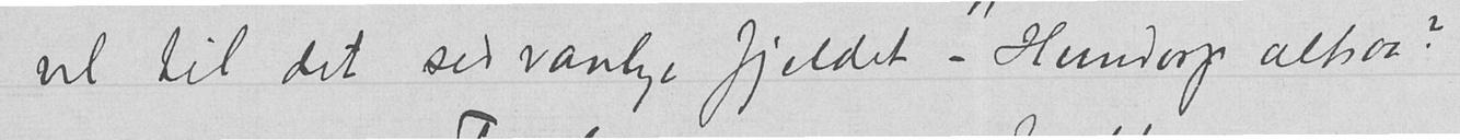vel til det selvanlige fjeldet – Hundorp altsaa?
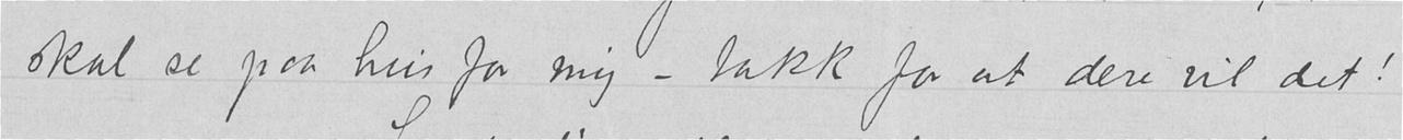skal se paa hus for mig – takk for at dere vil det!
